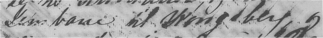Jernbane til Kongsberg, og
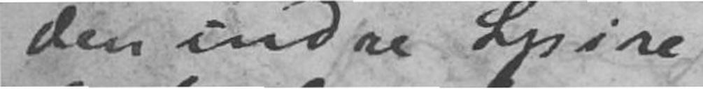den indre Spire
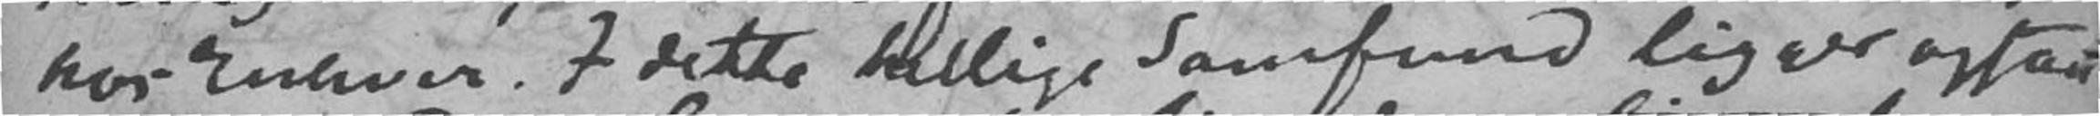hos Enhver. I dette hellige Samfund ligger ogsaa
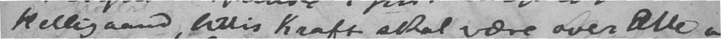Helligaand, hvis Kraft skal være over alle og
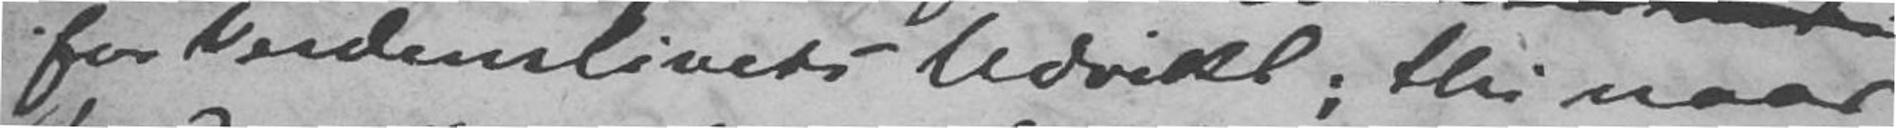for Verdenslivets Udvikl; thi naar
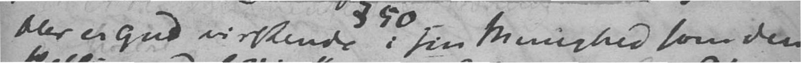Mer er Gud virkende i sin Menighed som den
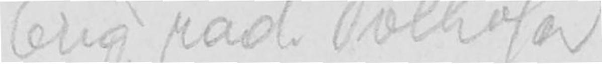Orig. rad. Tælhøfe/Tolhof
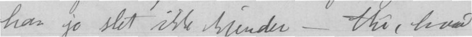han jo slet ikke kjender - thi, hvad
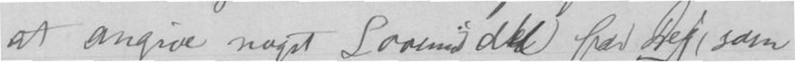at angive noget Sovemiddel for Dig, som
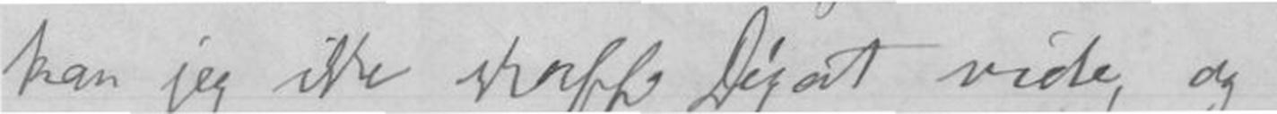kan jeg ikke skaffe Dig at vide, og
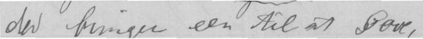der bringer en til at Sove,
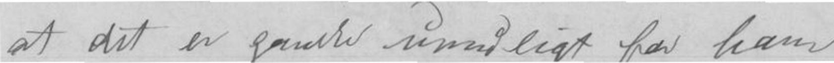at det er ganske umuligt for ham
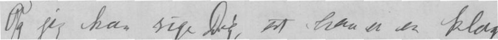Og jeg kan sige Dig, at han er en klog,
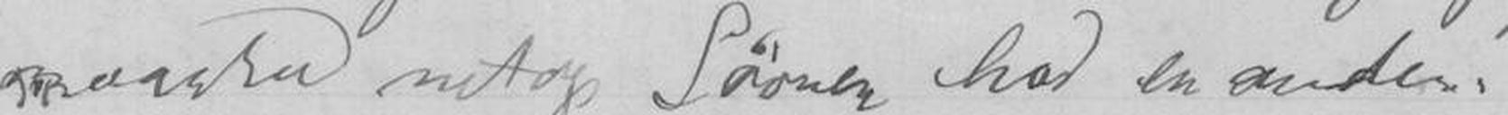maaske netop Søvnen hos en anden.
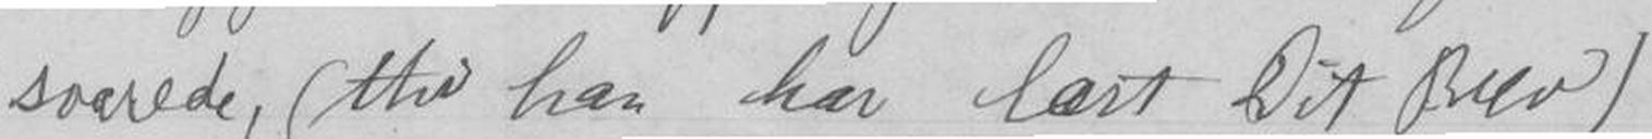svarede, (thi han har læst Dit Brev)
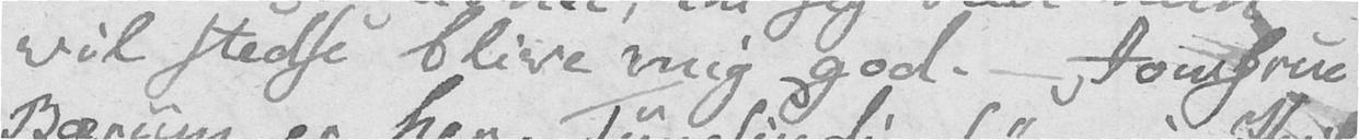vil stedse blive mig god. – Jomfrue
